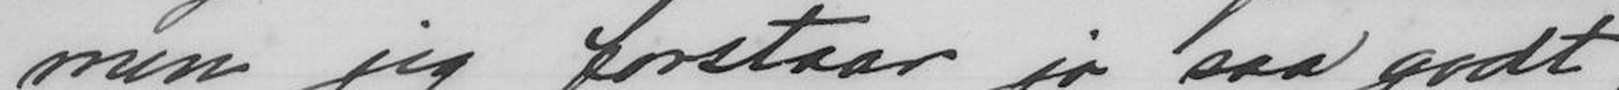men jeg forstaar jo saa godt,
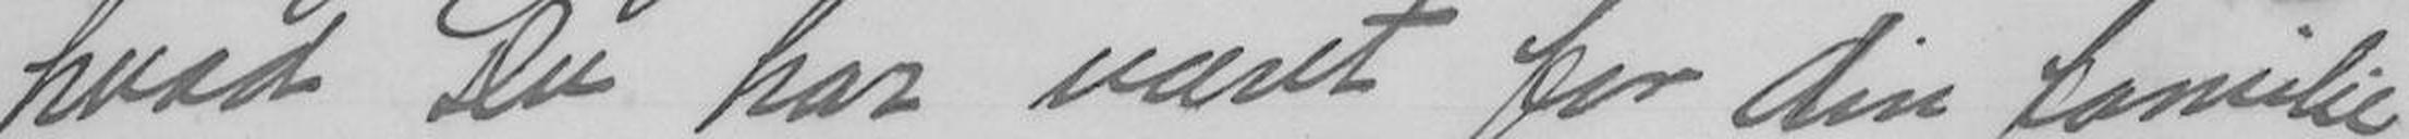hvad Du har været for din familie
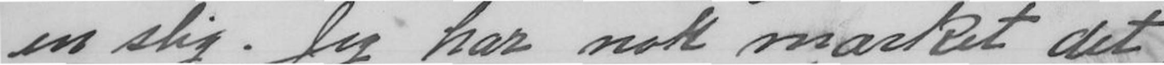en slig. Jeg har nok mærket det,
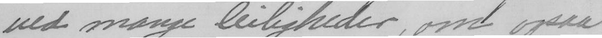ved mange leiligheder, om ogsaa
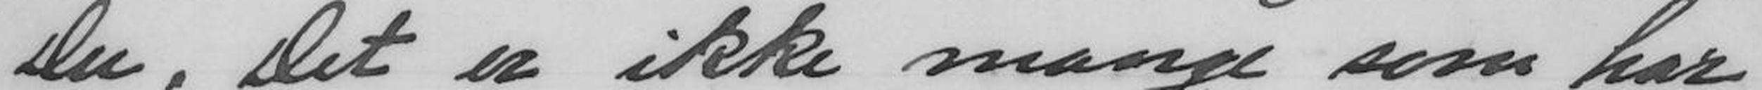Du. Det er ikke mange som har
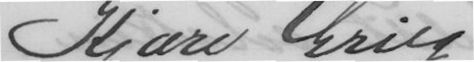Kjære Grieg
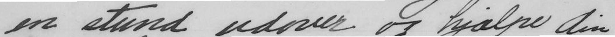en stund udover og hjælpe din
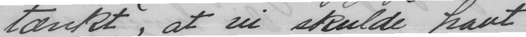tænkt, at vi skulde havt
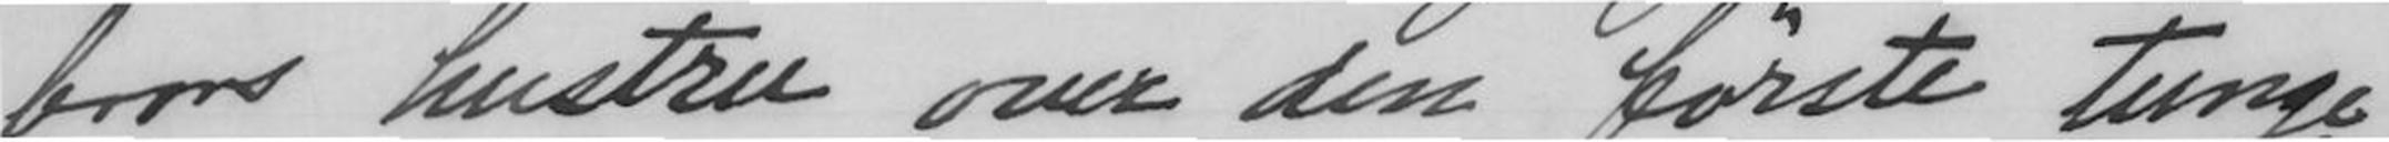brors hustru over den første tunge
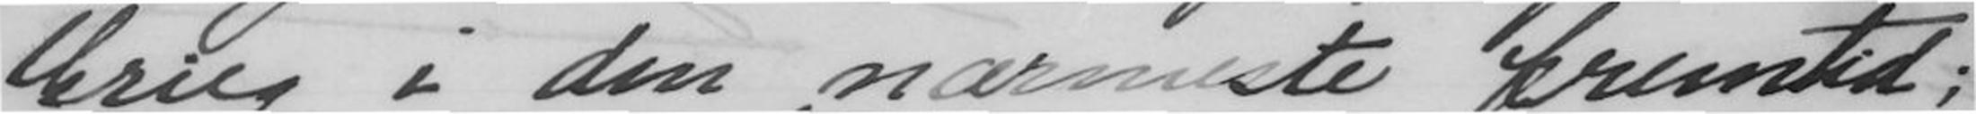Grieg i den nærmeste fremtid;
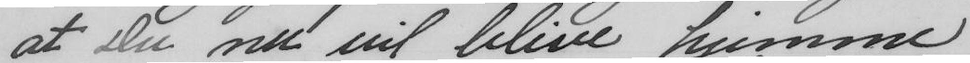at Du nu vil blive hjemme
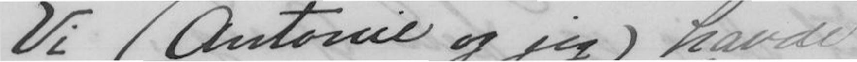Vi (Antonie og jeg) havde
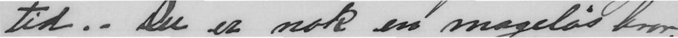tid. - Du er nok en mageløs bror,
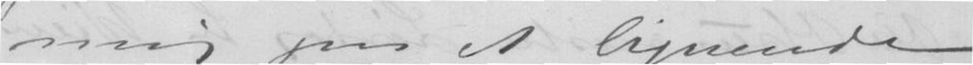mig paa et lignende
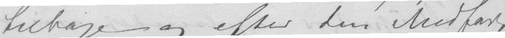tilbage og efter den Medfart
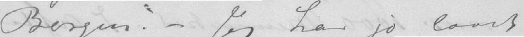Bergen. - Jeg har jo lovet
